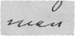man
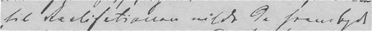til Realisationen vilde de frembyde
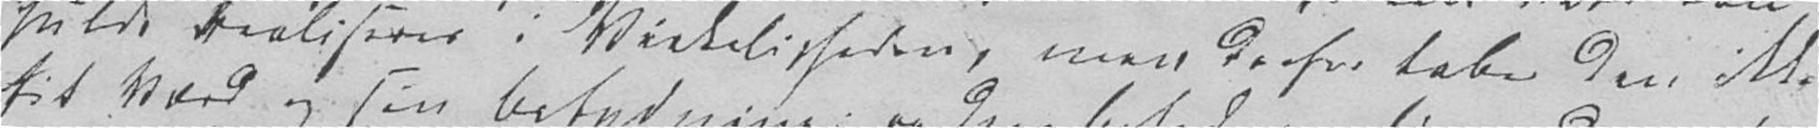fuldt realiseres i Virkeligheden, men derfor taber den ikke
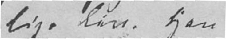lige Liv. Han
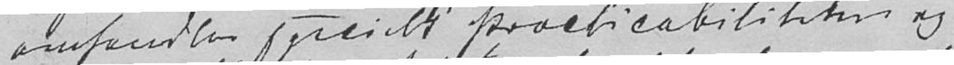omhandler specielt Practicabiliteten og
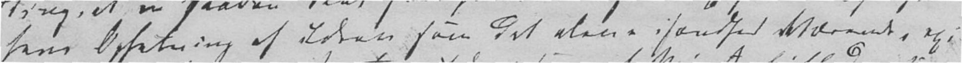hans Opfatning af Ideen som det alene isandhed Værende, exi-
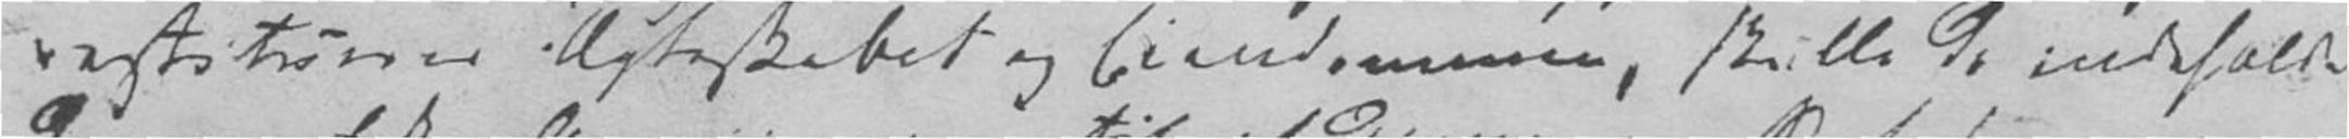restituerer Ægteskabet og Eiendommen, skulle da indeholde
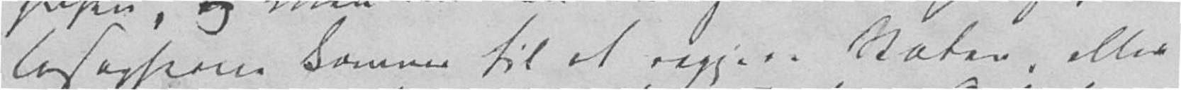losopherne kommer til at regjere Staten,
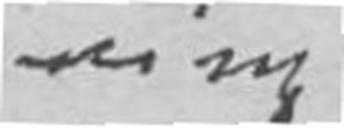ning
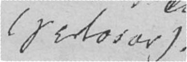(doror).
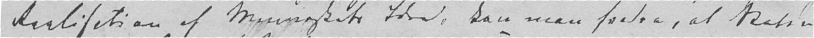Realisation af Menneskets Idee, kan man fordre, at Staten
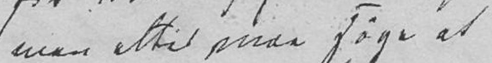man altid maa søge at
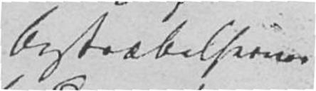Bestræbelserne
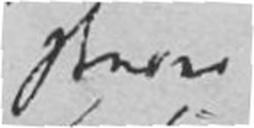sterer
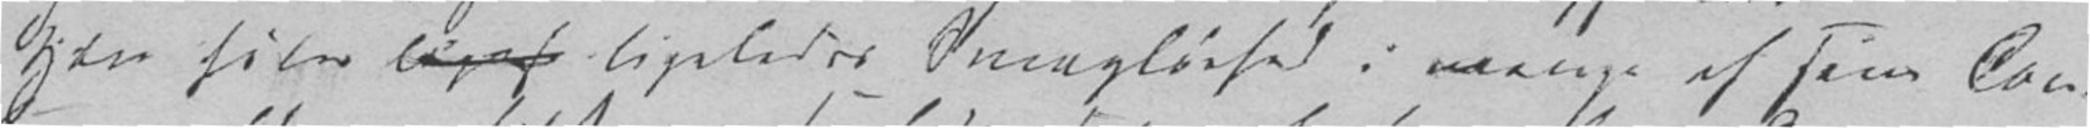Han føler ligel ligeledes Smagløshed i mange af sine Con-
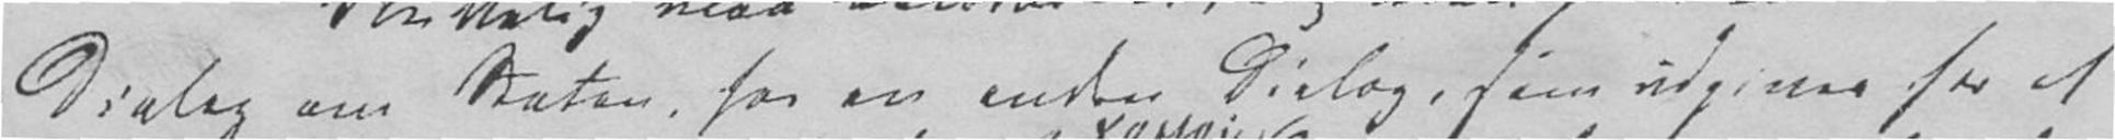Dialog om Staten, har en anden Dialog, som udgives for at
